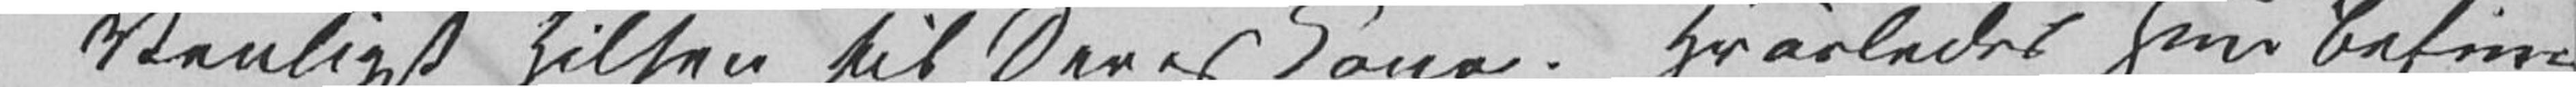Venligst Hilsen til Deres Kone. Hvorledes hun befin¬
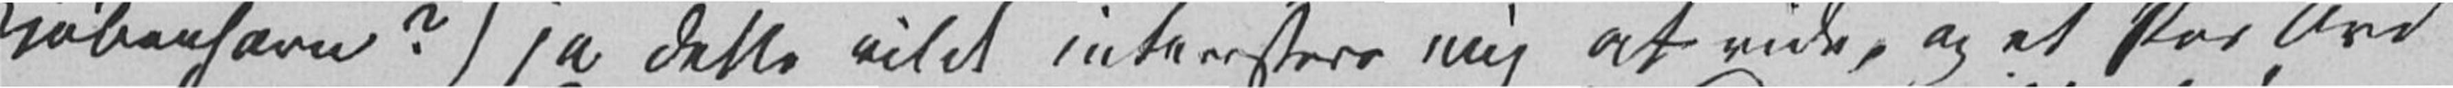Kjøbenhavn?) ja dette vilde interessere mig at vide, og et Par Ord
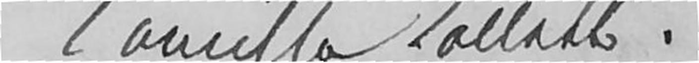Camilla Collett.
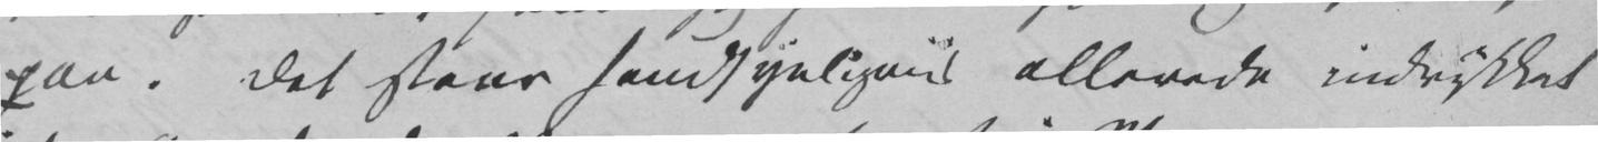paa. Det staar sandsynligviis allerede indrykket
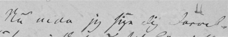Nu maa jeg sige Dig Farvel –
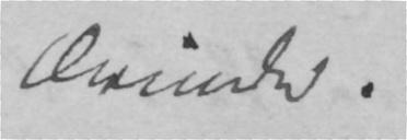Ærinder.
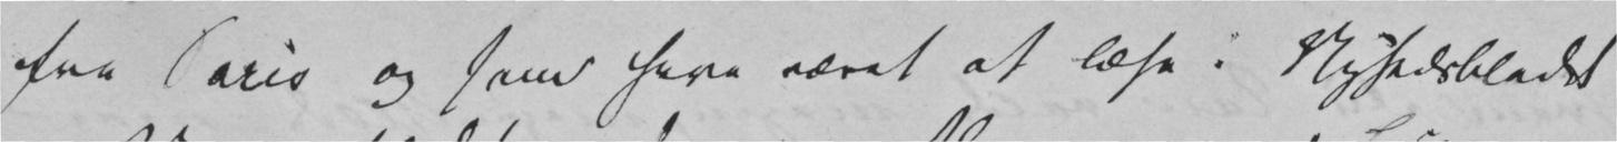fra Paris og som have været at læse i Nyhedsbladet
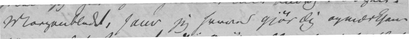Morgenbladet, som jeg herved gjør Dig opmærksom
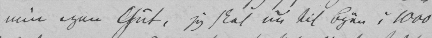min egen Gut, jeg skal nu til Byen i 1000
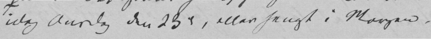idag Onsdag den 23de, eller senest i Morgen.
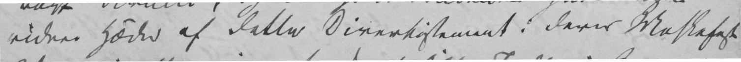videre Hæder af dette Divertissement i deres Maskefest
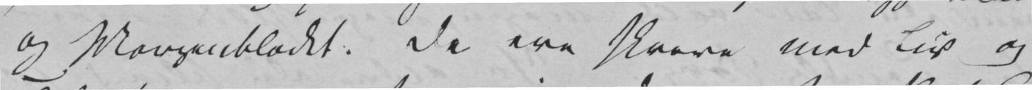og Morgenbladet. De ere skrevne med Liv og
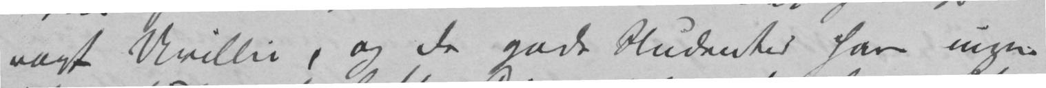vagt Uvillie, og de gode Studenter have ingen
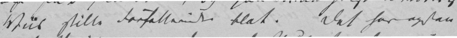Viis stille Forfatterinden blot. Det har ogsaa
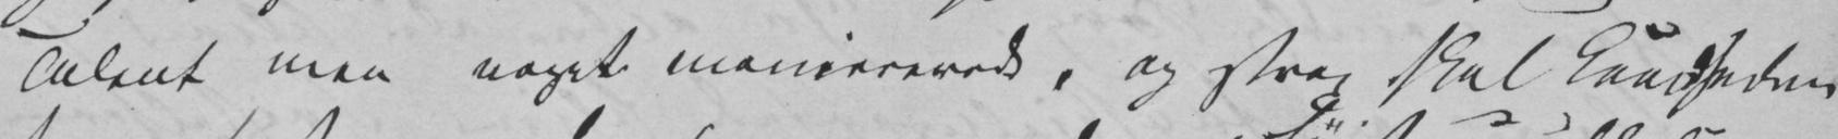Talent men noget maniereredt, og strax skal Kaadheden
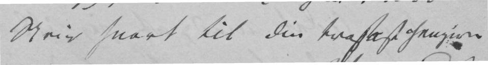Skriv snart til Din trofaste hengivne
